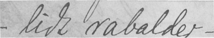- lidt rabalder -
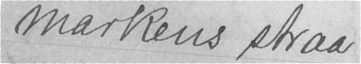markens straa
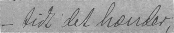- tidt det hænder,
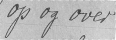op og over
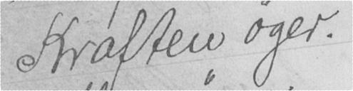Kraften øger.
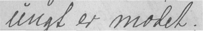ungt er modet;
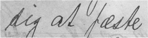sig at fæste
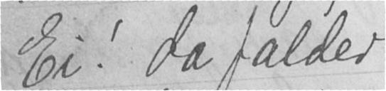Ei! da falder
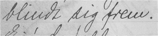blindt sig frem.
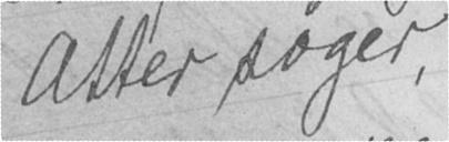Atter søger,
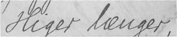- Higer længer,
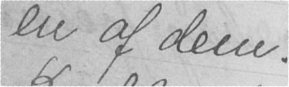en af dem.
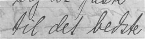til det bedste
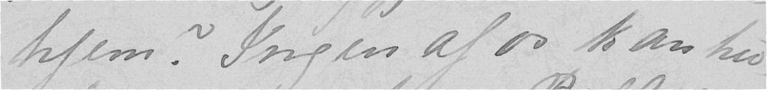hjem? Ingen af os kan hu-
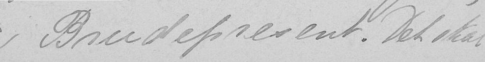i Brudepresent. Det skal
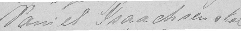Daniel. Isaachsen skal
